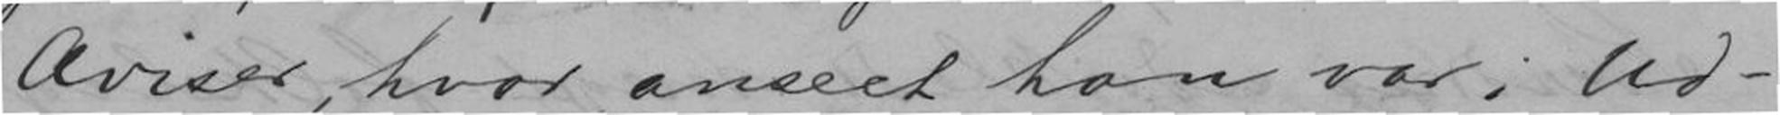Aviser, hvor anseet han var i Ud-
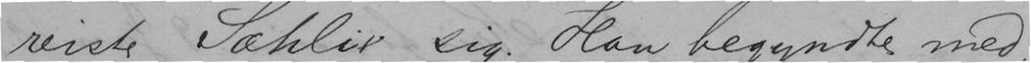reiste Sæhlir sig. Han begyndte med
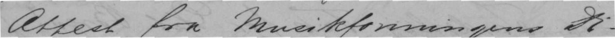Attest fra Musikforeningens Di-
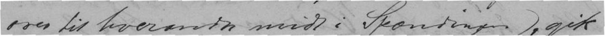over til hverandre midt i Spændingen), gik
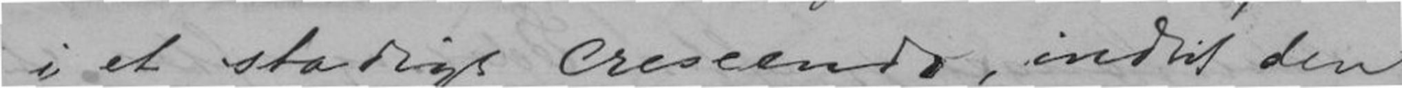i et stadigt crescendo, indtil den
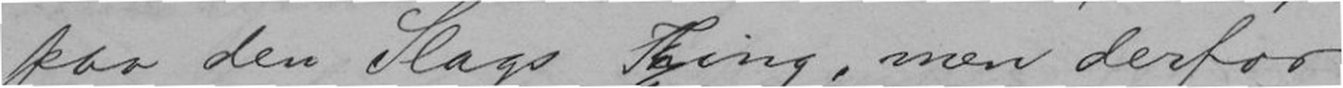paa den Slags Thing, men derfor
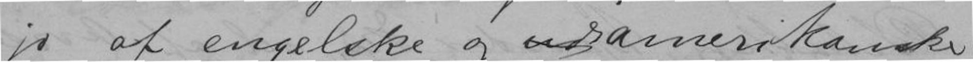jo af engelske og ud amerikanske
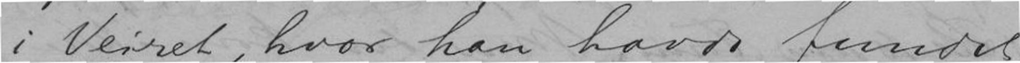i Veiret, hvor han havde fundet
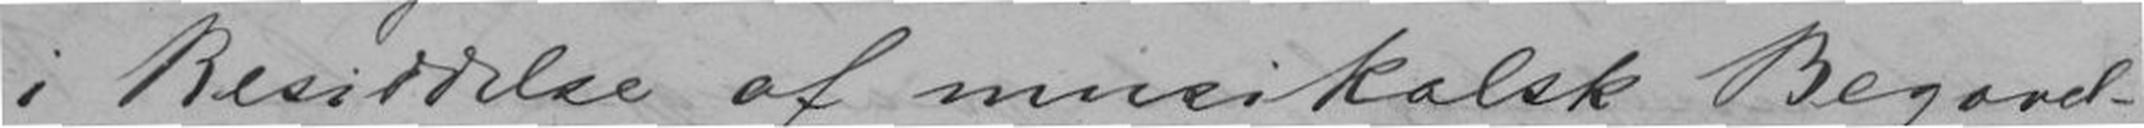i Besiddelse af musikalsk Begavel-
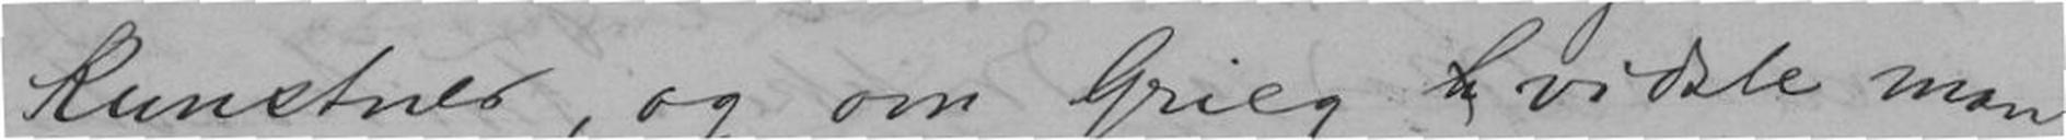Kunstner, og om Grieg h vidste man
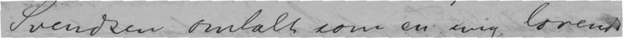Svendsen omtalt som en ung lovende
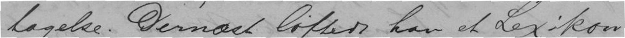tagelse. Dernæst løftede han et Lexikon
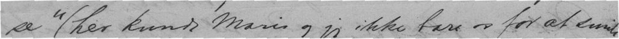se (her kunde Marie og jeg ikke bare os for at smile
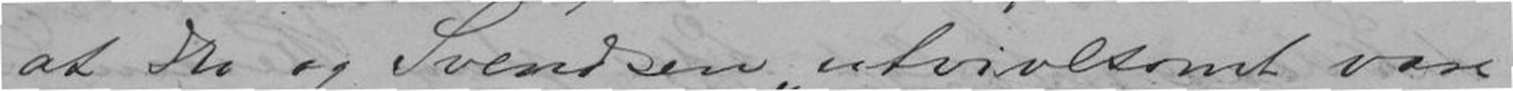at Du og Svendsen utvivlsomt være
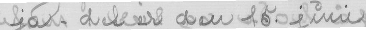ja - det er den 15 juni
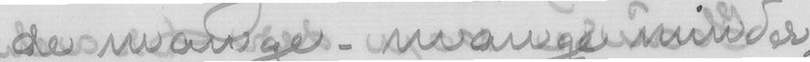de mange - mange minder.
